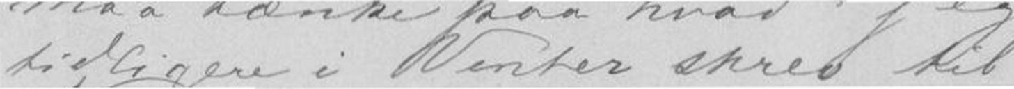tidligere i Vinter skrev til
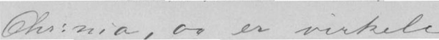Chr:nia, og er virkelig
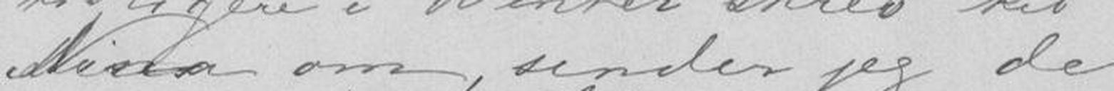Nina om, sender jeg de
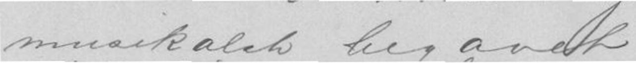musikalsk begavet. -
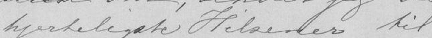hjerteligste Hilsener til
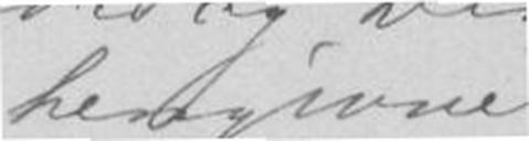hengivne
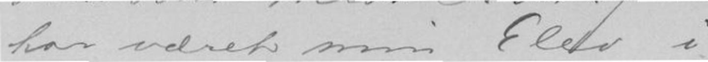har været min Elev i
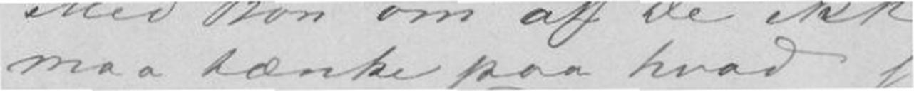maa tænke paa hvad jeg
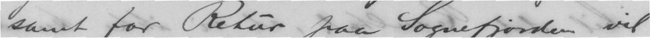samt for Retur paa Sognefjorden vil
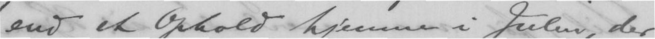end et Ophold hjemme i Julen, der
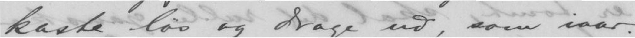kaste løs og drage ud, som iaar.
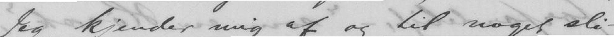Jeg kjender mig af og til noget sli-
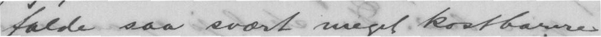falde saa svært meget kostbarere
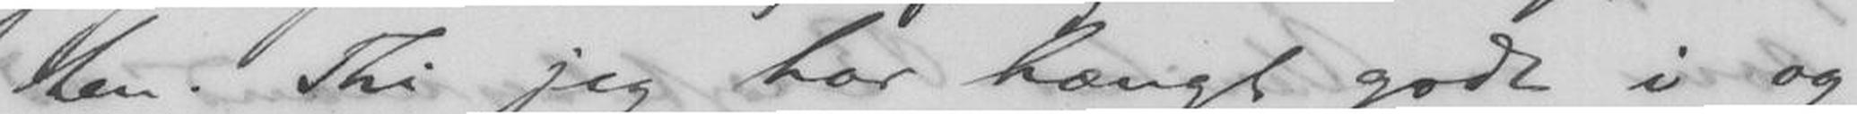ten. Thi jeg har hængt godt i og
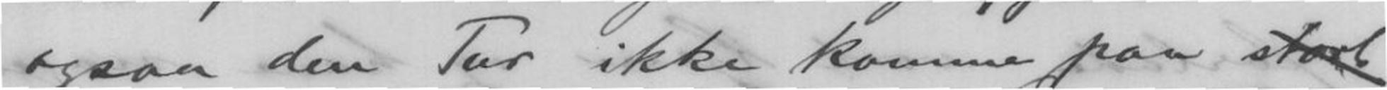ogsaa den Tur ikke komme paa stort
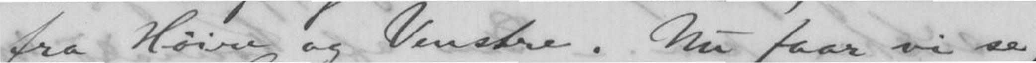fra Høire og Venstre. Nu faar vi se,
